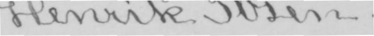Henrik Ibsen.
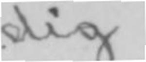dig.
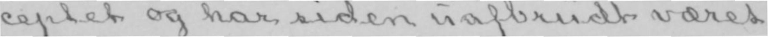ceptet og har siden uafbrudt været
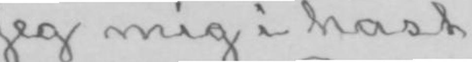jeg mig i hast
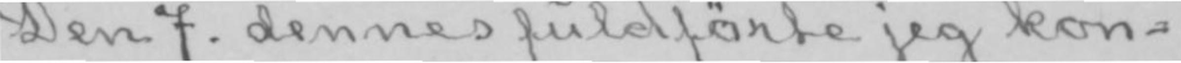Den 7. dennes fuldførte jeg kon-
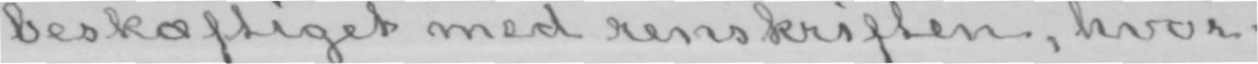beskæftiget med renskriften, hvor-
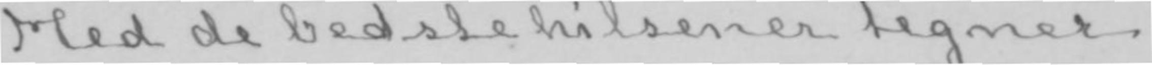Med de bedste hilsener tegner
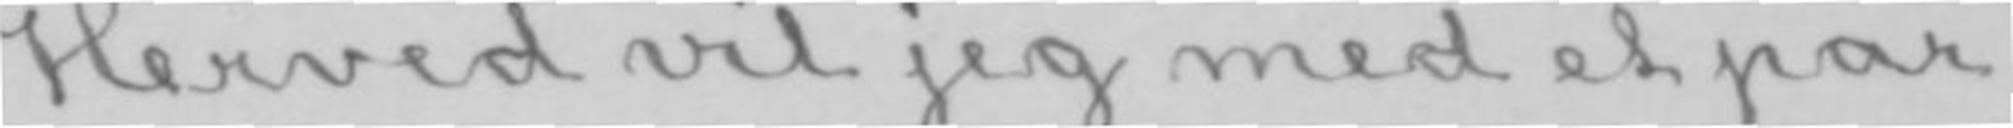Herved vil jeg med et par
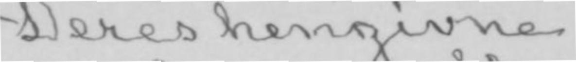Deres hengivne
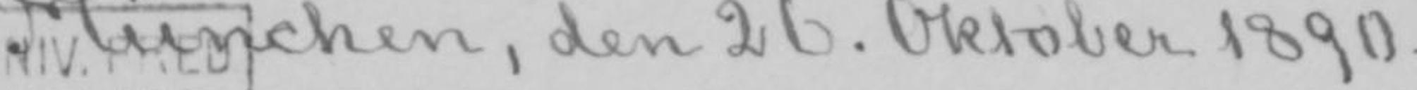München, den 26. Oktober 1890.
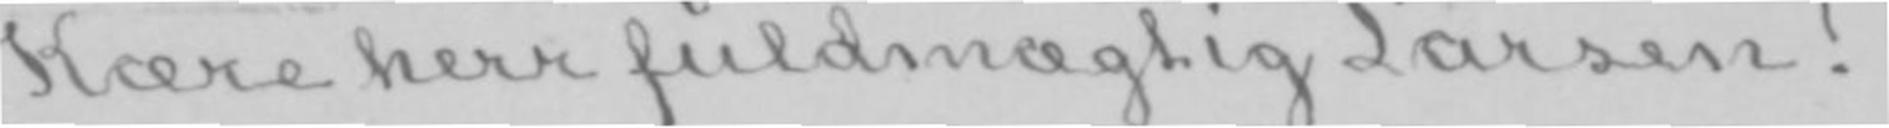Kære herr fuldmægtig Larsen!
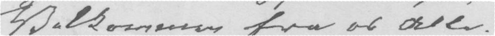Velkommen fra os Alle.
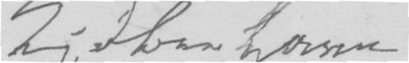Kjøbenhavn
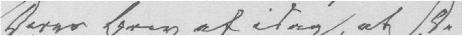Deres Brev af idag, at De
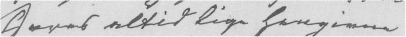Deres altid lige hengivne
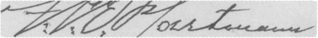J.P.E.Hartmann
